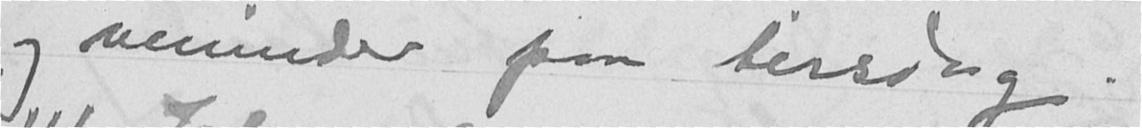og veninder paa tirsdag.
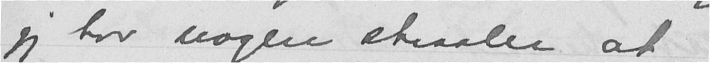jeg har nogen straaler at
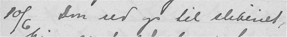10/6 Don red op til sliberiet,
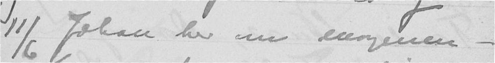11/6 Johan her om morgenen -
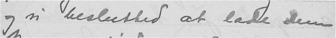og vi beslutted at lade dem
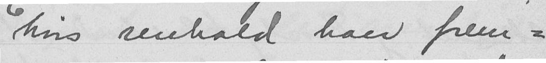hvis renhold han frem-
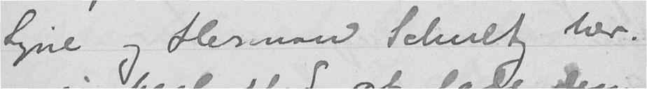Signe og Herman Schultz her.
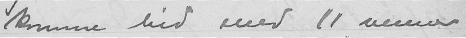komme hid med 11 venner
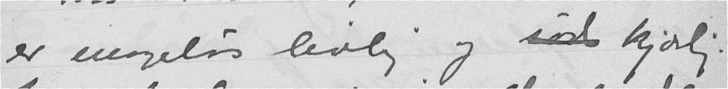er mageløs livlig og sød kjærlig.
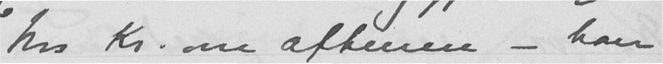hos Kr. om aftenen - han
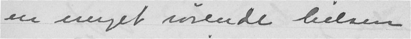en meget rørende hilsen
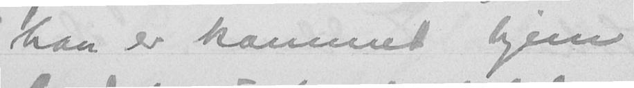han er kommet hjem
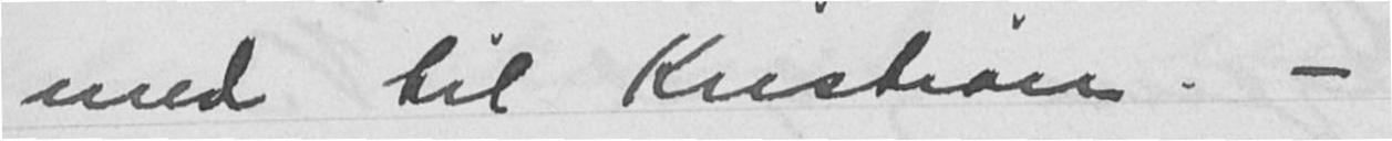med til Kristian. -
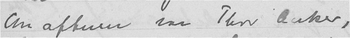Om aftenen var Thor Anker,
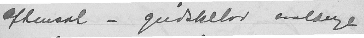aftenssol - gudskelov saalænge
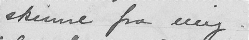skinne fra mig.
